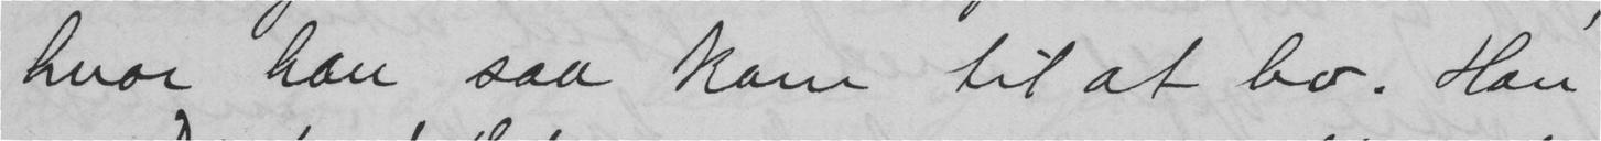hvor han saa kom til at bo. Han
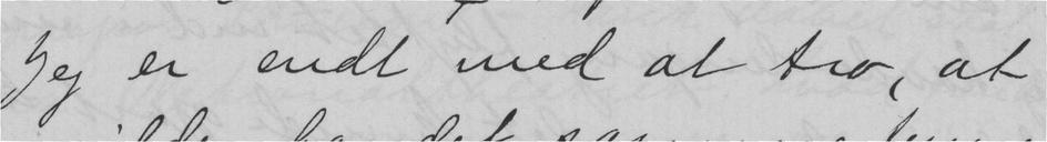Jeg er endt med at tro, at
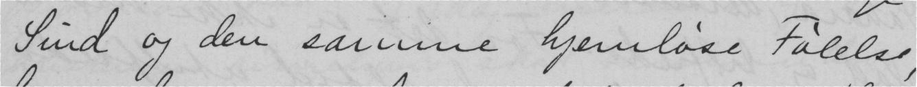Sind og den samme hjemløse Følelse,
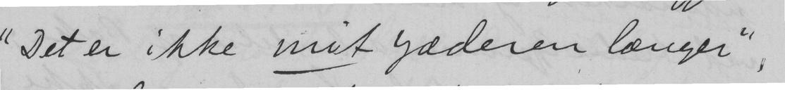"Det er ikke mit Jæderen længer",
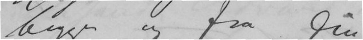begge og fra Din
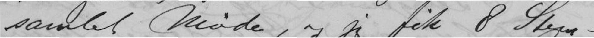samlet Møde, og jeg fik 8 Stem-
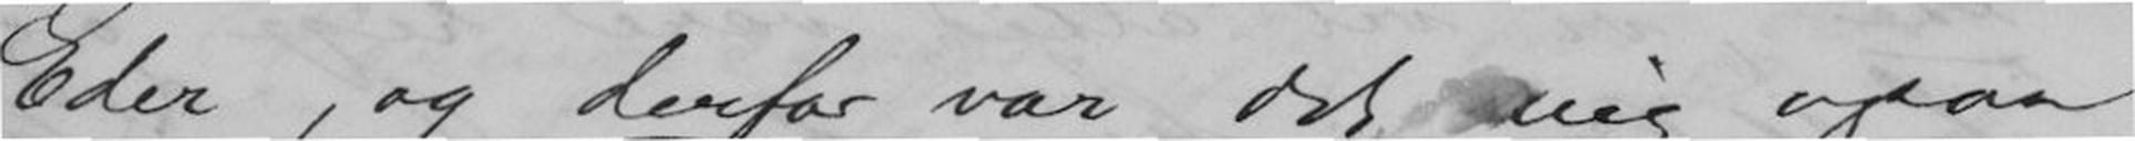Eder, og derfor var det mig ogsaa
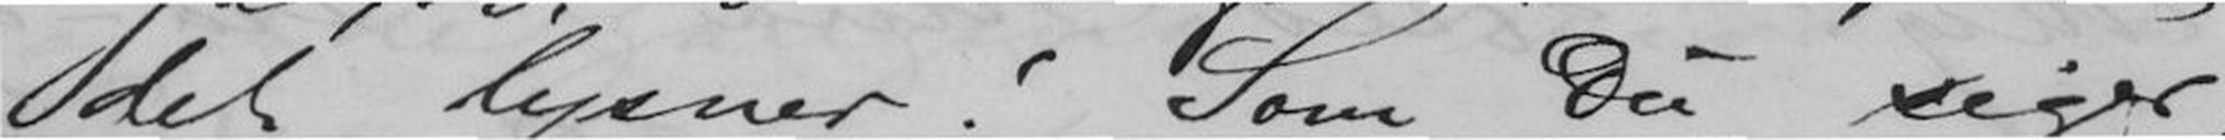det lysner! Som Du siger,
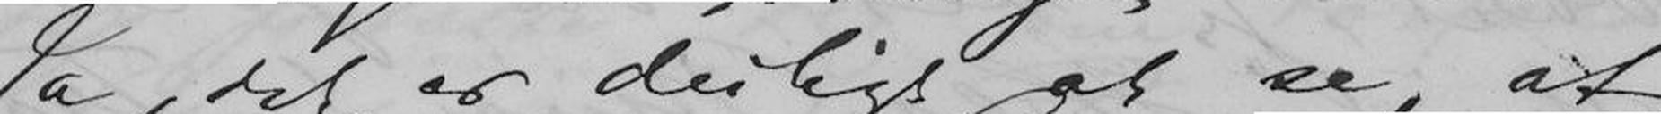Ja, det er deiligt at se, at
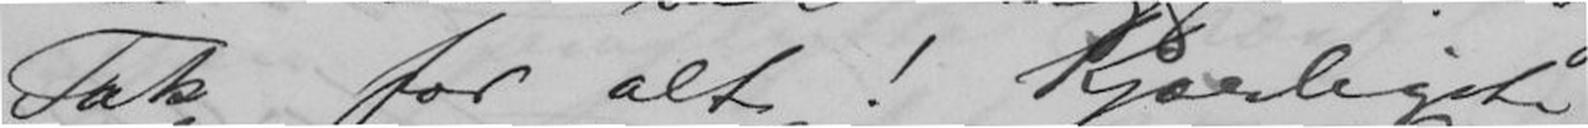Tak for alt! Kjærligste
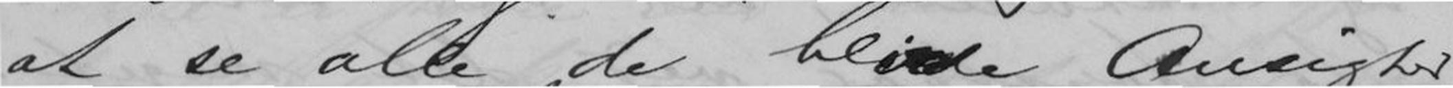at se alle de blide Ansigter,
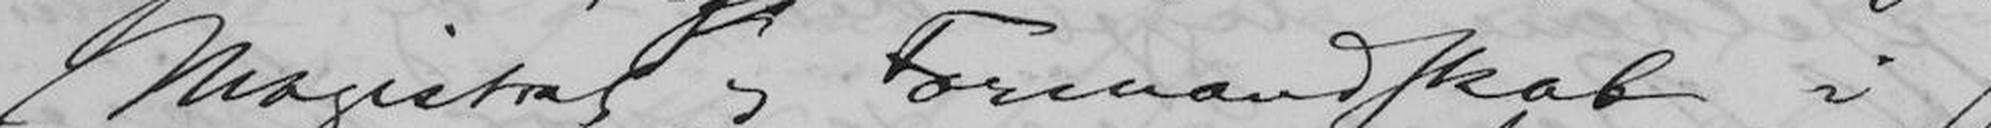Magistrat og Formandskab i
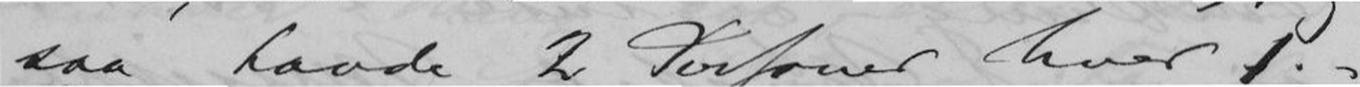saa havde 2 Personer hver 1.
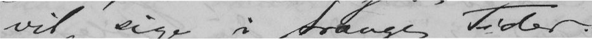vil sige i trange Tider.
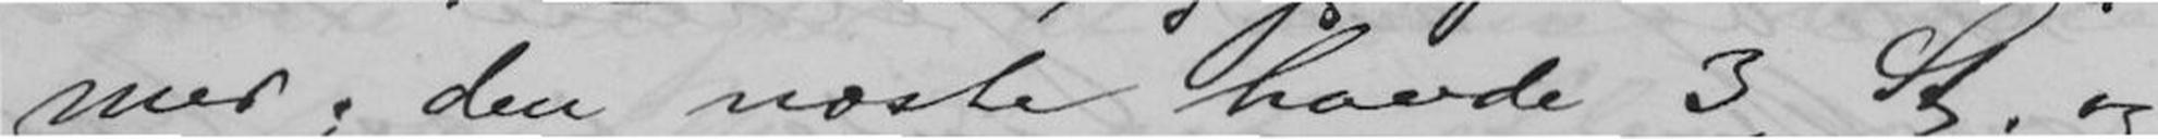mer, den næste havde 3 St. og
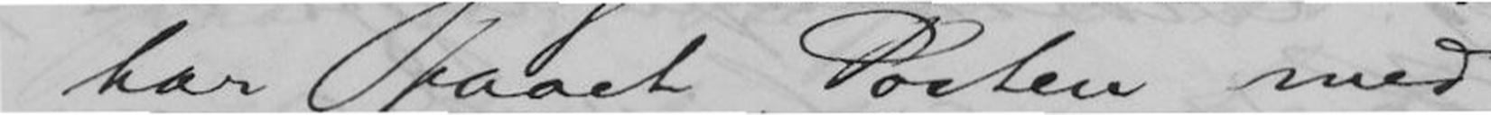jeg har faaet Posten med
